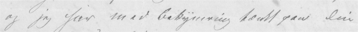og jeg har med Bekymring tænkt paa Din
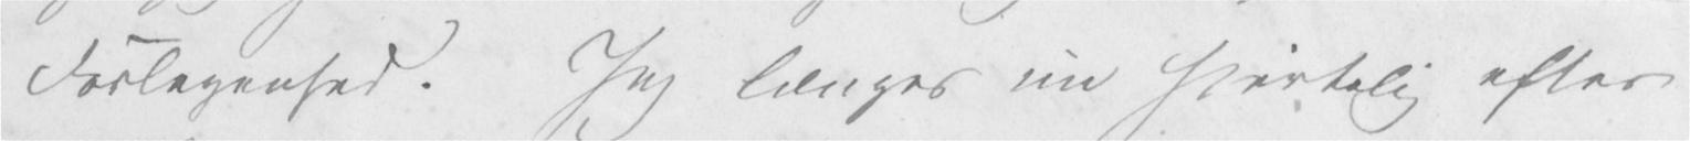Forlegenhed. Jeg længes nu hjertelig efter
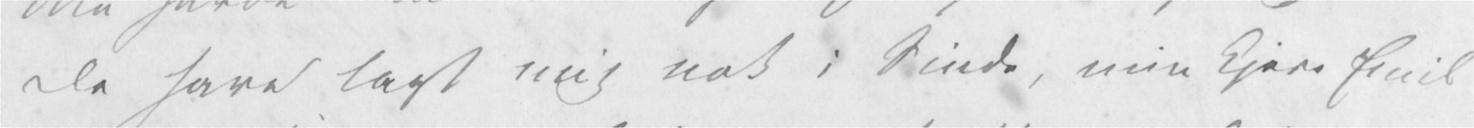De have lagt mig nok i Sinde, min kjere Emil
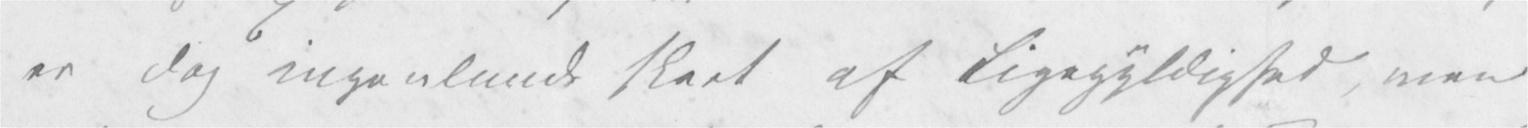er dog ingenlunde skeet af Ligegyldighed, men
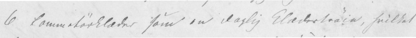6 Lommetørklæder som en daglig Klædestrøie, hvilket
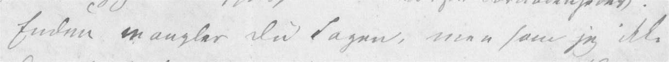Endnu mangler Du Lagen, men som jeg ikke
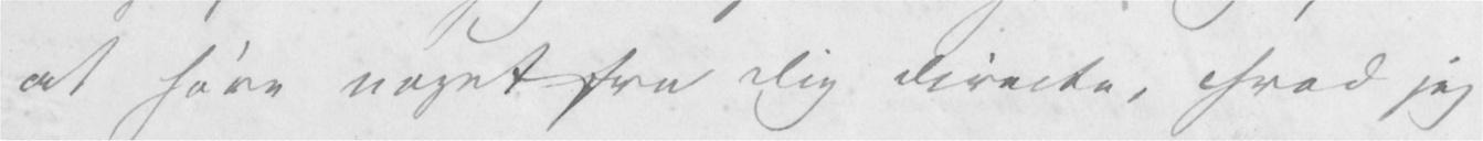at høre noget fra Dig directe, hvad jeg
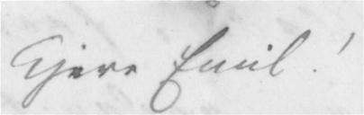Kjere Emil!
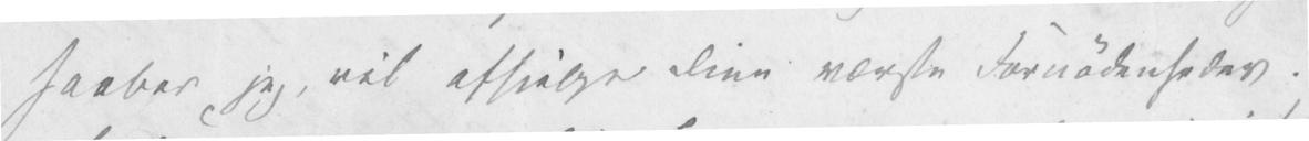haaber jeg, vil afhjelpe Dine værste Fornødenheder.
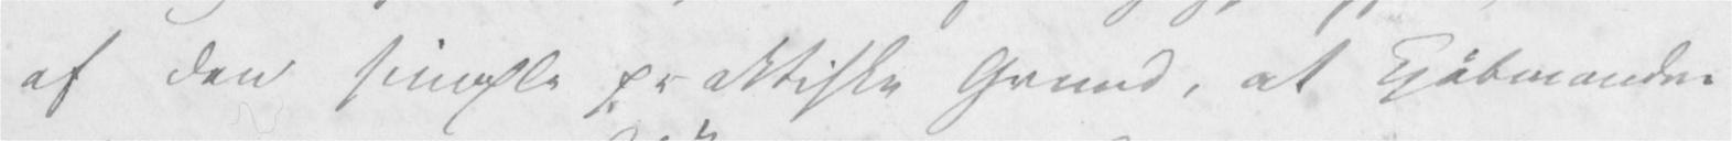af den simple praktiske Grund, at Kjøbmanden
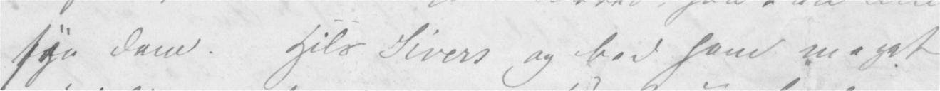sye dem. Hils Sivers og bed ham meget
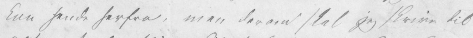kan sende herfra, men derom skal jeg skrive til
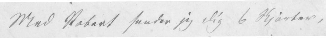Med Robert sender jeg Dig 6 Skjorter,
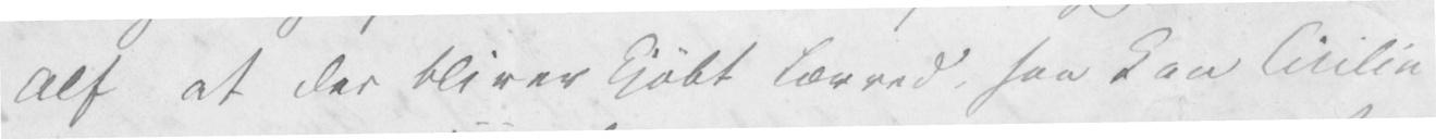Alf at der bliver kjøbt Lærred, saa kan Cicilie
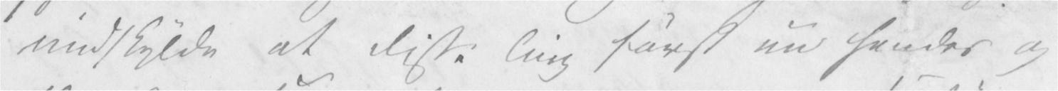undskylde at disse Ting først nu sendes og
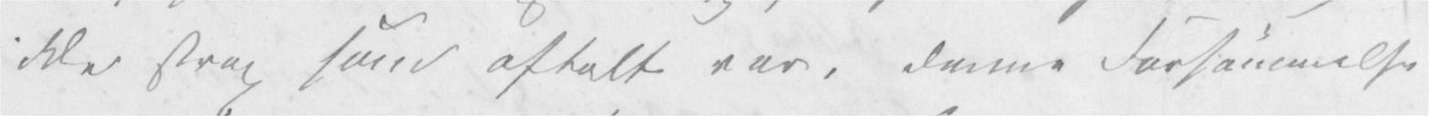ikke strax som aftalt var, denne Forsømmelse
